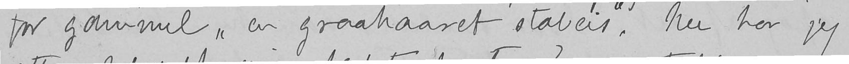for gammel "en graahaaret stabeis". Nu har jeg
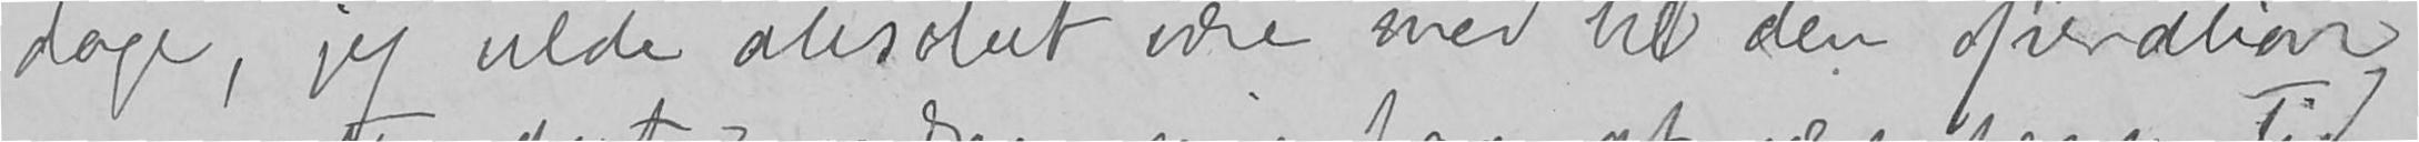dage, jeg vilde absolut være med til den operation
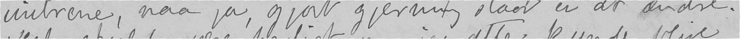vintrene, naa ja, gjort gjerning staar ei at ændre.
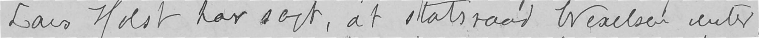Lars Holst har sagt, at statsraad Wexelsen venter,
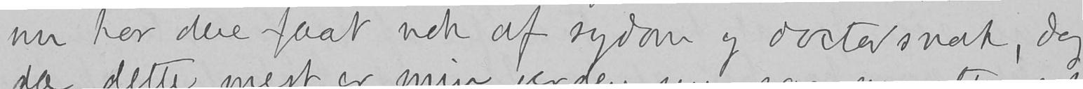nu har dere faat nok af sygdom og doctorsnak, dog
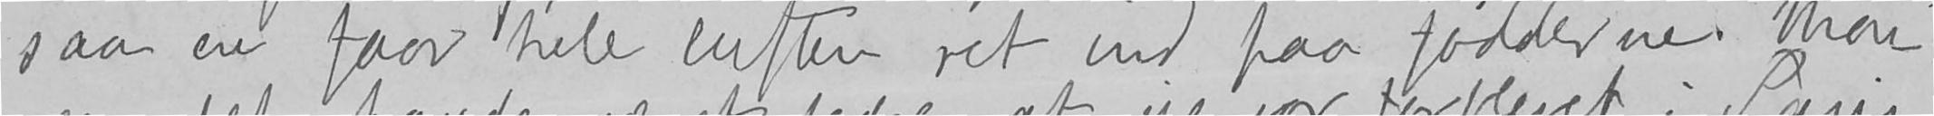saa en faar hele luften ret ind paa fødderne. Mon
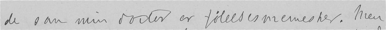de som min doctor er følelsesmennesker. Men
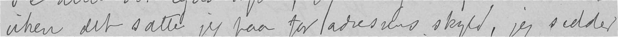viken, det satte jeg paa for adressens skyld, jeg sidder
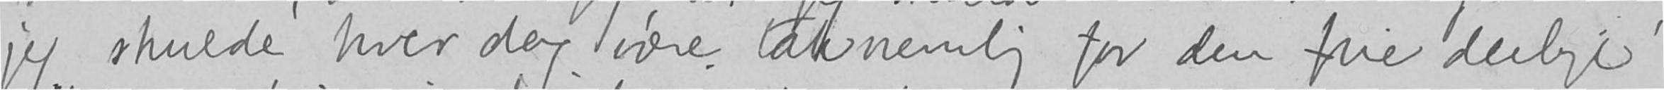jeg skulde hver dag være taknemlig for den frie deilige
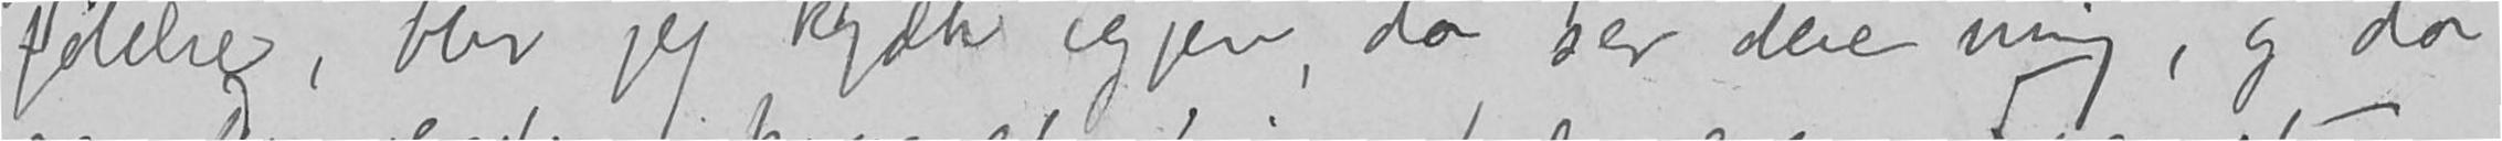følelse, blir jeg kjæk igjen da ser dere mig, og da
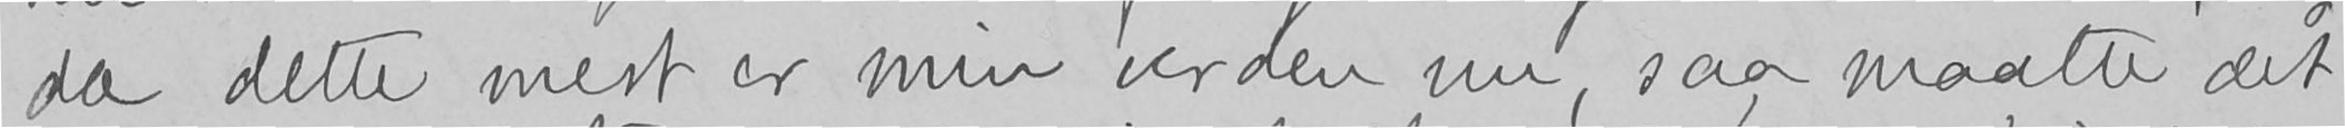da dette mest er min verden nu, saa maatte det
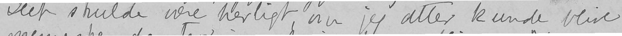Det skulde være herligt om jeg atter kunde blive
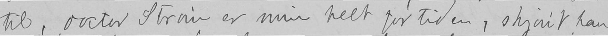til, doctor Strøm er min helt for tiden, skjønt han
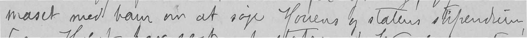maset med ham om at søge Houens og statens stipendium,
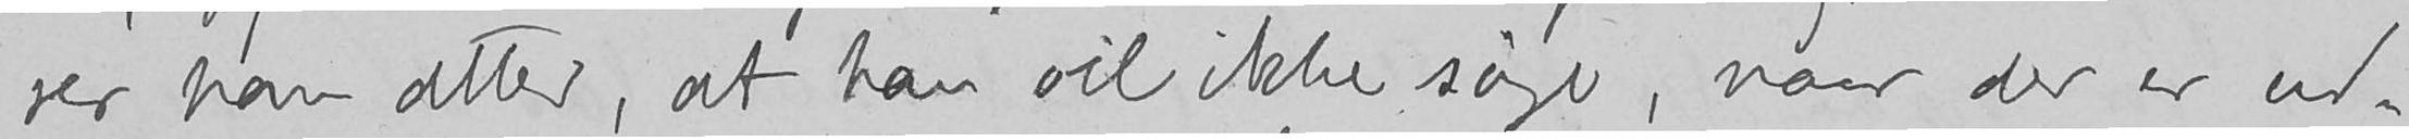rer han atter, at han vil ikke søge, naar der er ud-
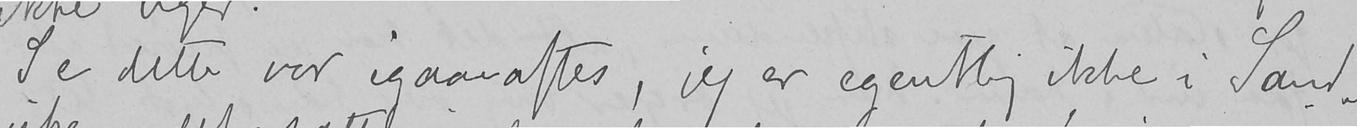Se dette var igaaraftes, jeg er egentlig ikke i Sand-
